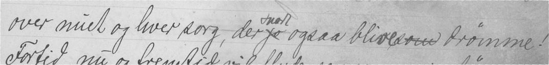over nuet og hver sorg, der snart ogsaa blive som drømme!
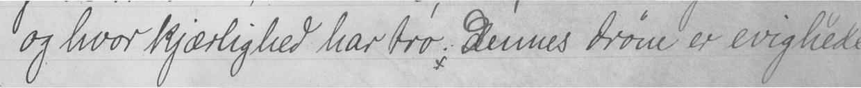og hvor kjærlighed har tro. ; Dennes drøm er evighede
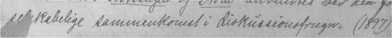selskabelige sammenkomst i diskussionsfrngn. (1897)
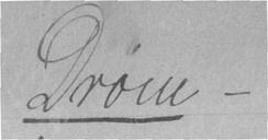Drøm -
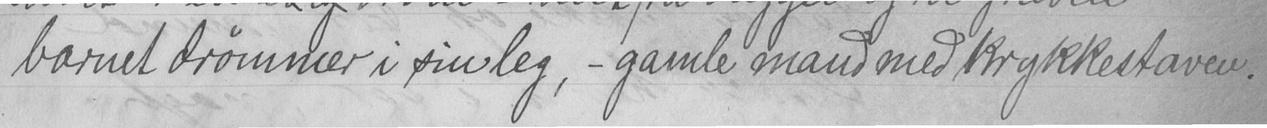barnet drømmer i sin leg, - gamle mand med krykkestaven.
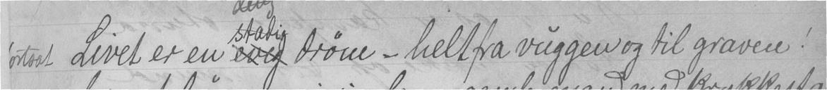tsat Livet er en evig drøm - helt fra vuggen og til graven!
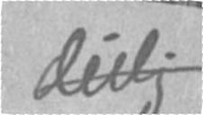deilj
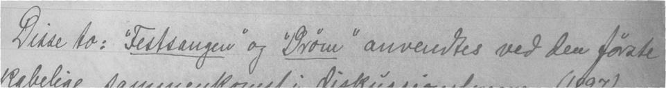Disse to: "Festsangen" og "Drøm" anvendtes ved den første
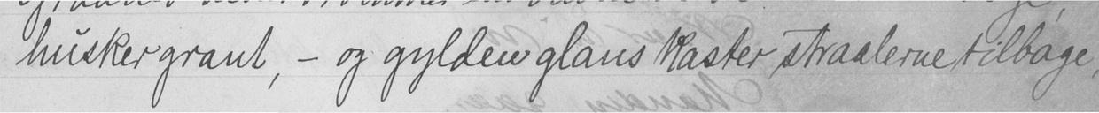husker grant, - og gylden glans kaster straalerne tilbage,
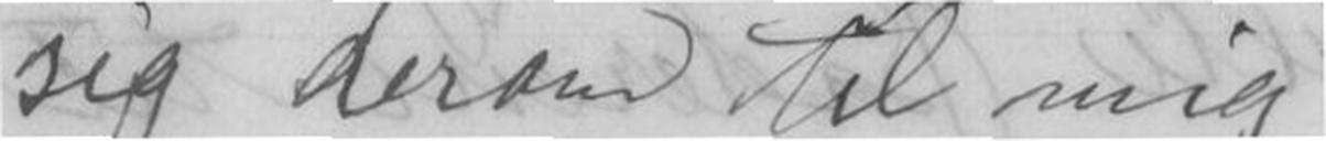sig derom til mig.
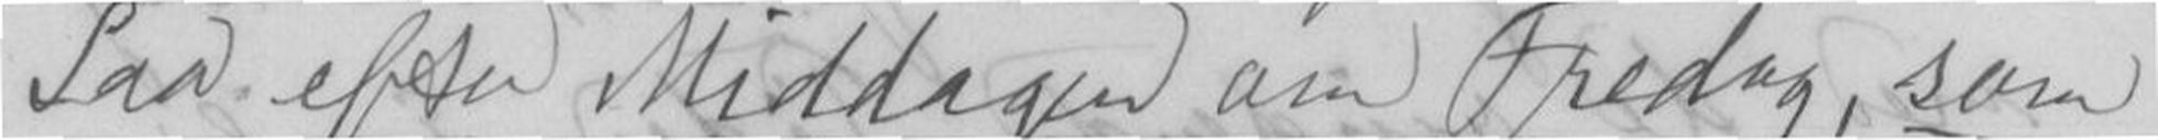Saa efter Middagen om Fredag, som
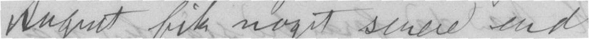August fik noget senere end
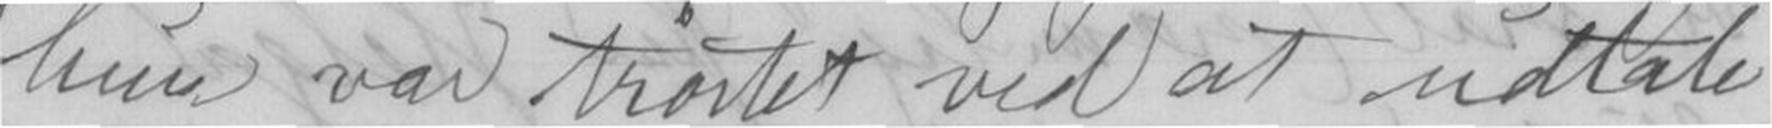hun var trøstet ved at udtale
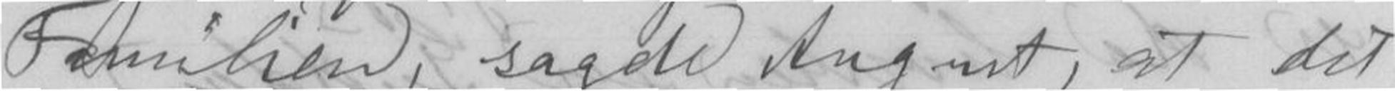Familien, sagde August, at det
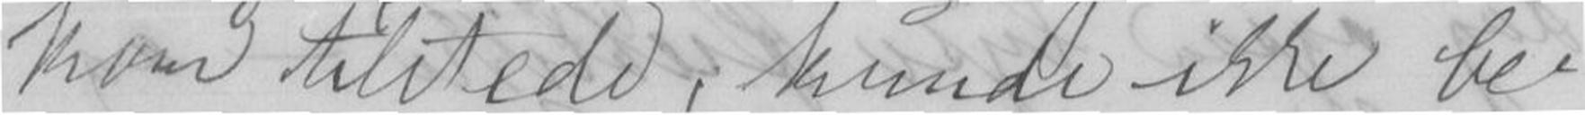kom tilstede, kunde ikke be-
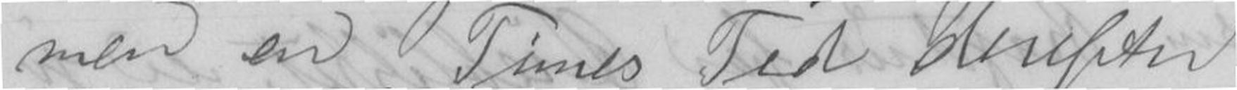men en Times Tid derefter
